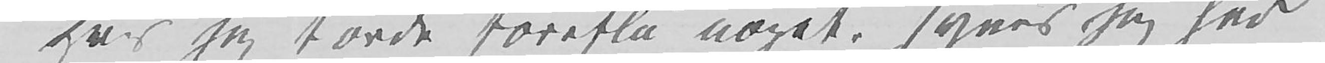Hvis jeg torde foreslå noget, synes jeg her
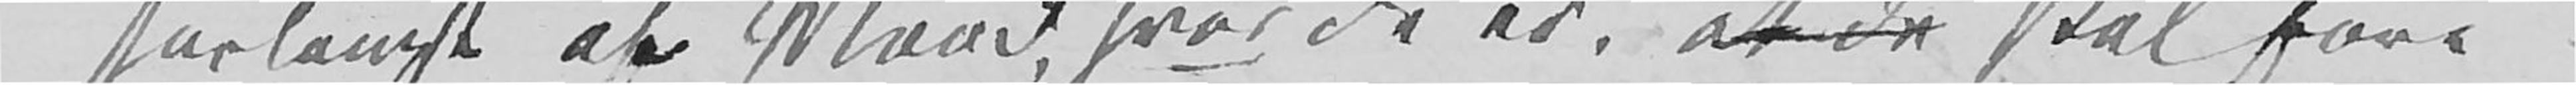forlangt at Mænd, hvor de er, at de skal føre
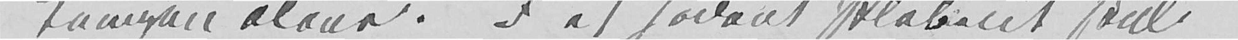Kampen alene. I et sådant Plebecit skal
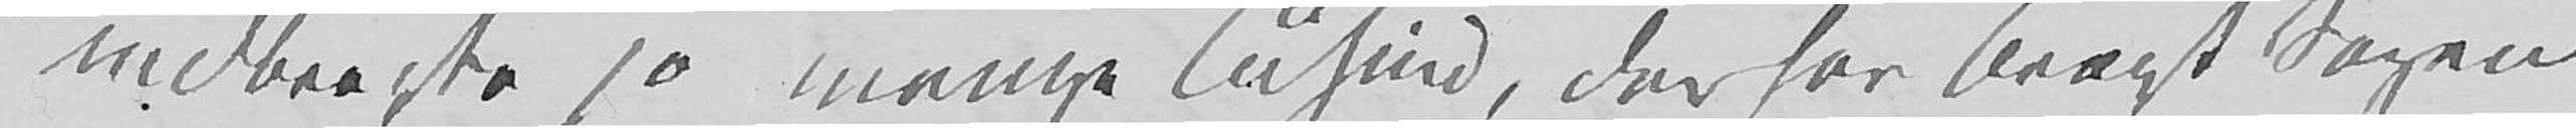indbragte jo mange Tusind, der har bragt Sagen
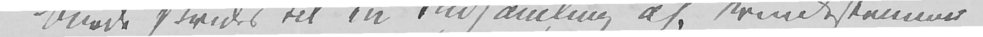burde skrides til en Indsamling af Kvindestemmer
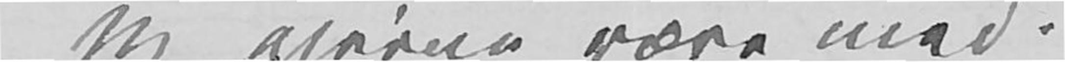jeg gjerne være med.
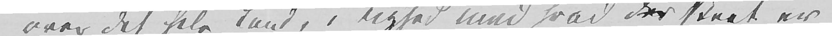over det hele Land, i Lighed med hvad der skeet er
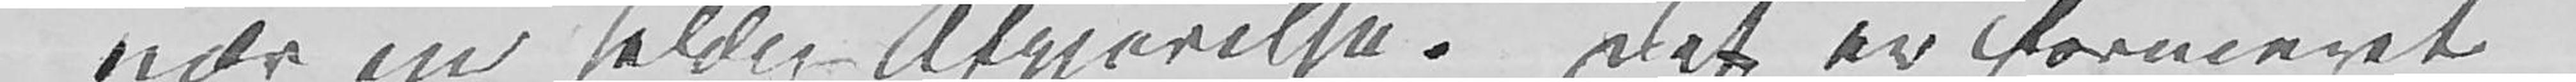nær en heldig Afgjørelse. Det er formeget
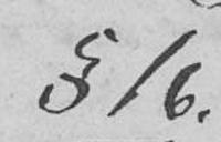§ 16.
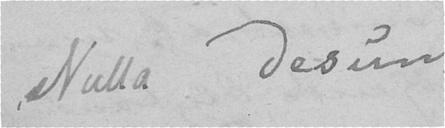Nulla Desunt
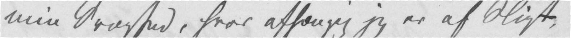min Svaghed, hvor afhængig jeg er af Sligt.
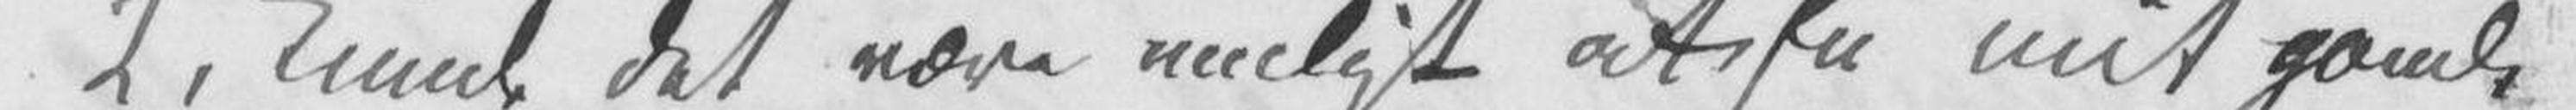2) Kunde det være muligt at få mit gamle
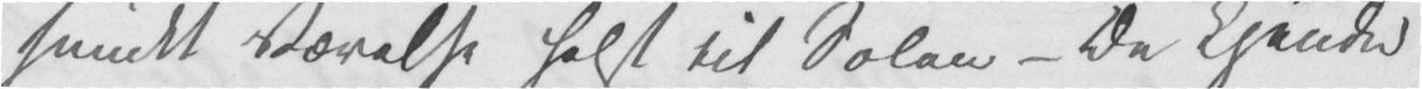smukt Værelse helst til Solen – De kjender
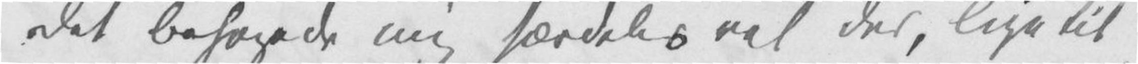Det behagede mig særdeles vel der, lige til
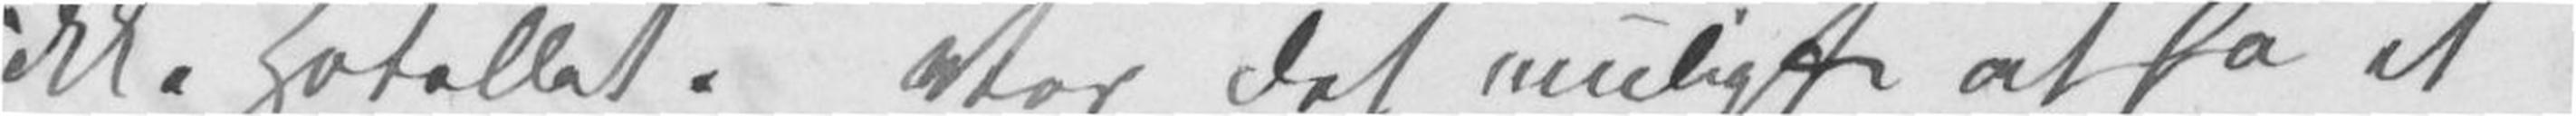ikke Hotellet? Var det muligt at få et
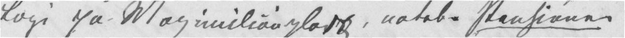Logi på Maximilianplatz, notab. Pensionen
